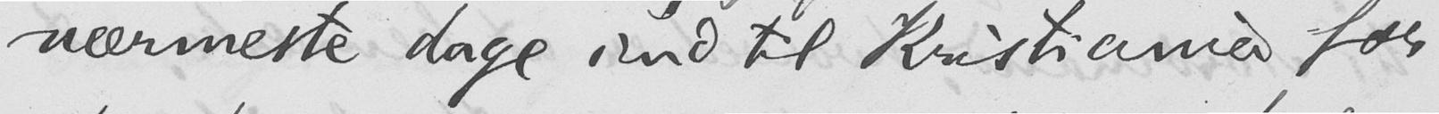nærmeste dage ind til Kristiania for
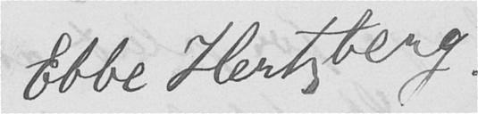Ebbe Hertzberg.
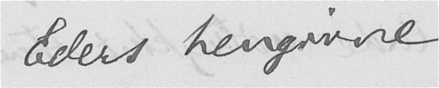Eders hengivne
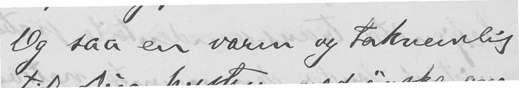Og saa en varm og taknemlig
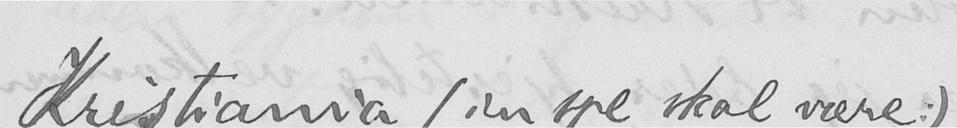Kristiania (in spe, skal være:)
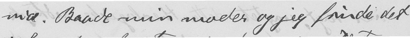nia. Baade min moder og jeg finde det
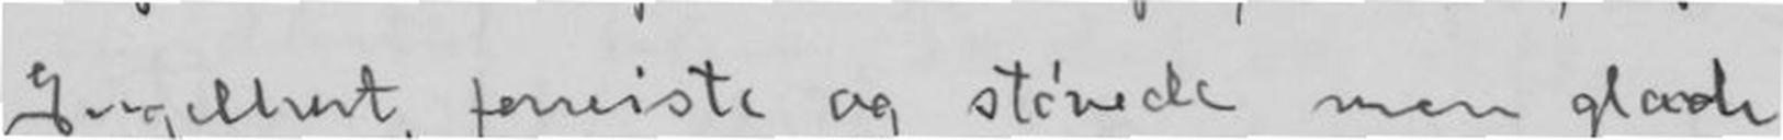Engelhart, forreiste og støvede men glade
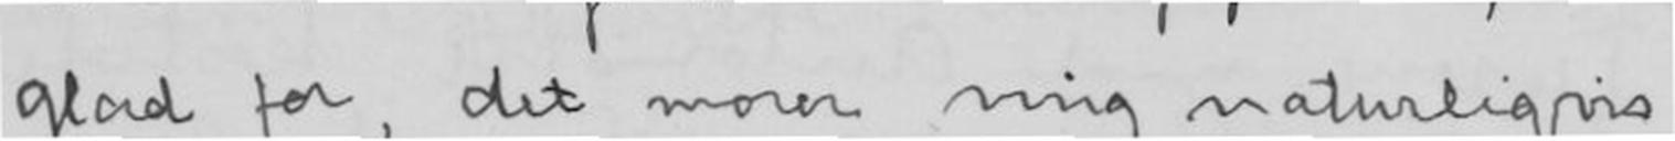glad for, det morer mig naturligvis
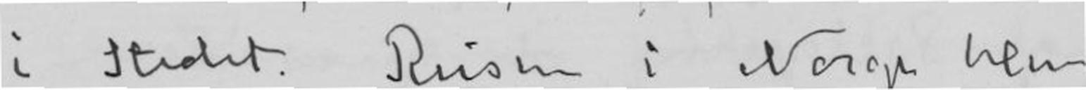i Stedet. Reisen i Norge blev
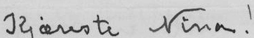Kjæreste Nina!
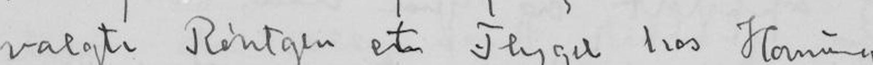valgte Röntgen et Flygel hos Homung
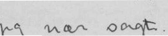jeg nær sagt.
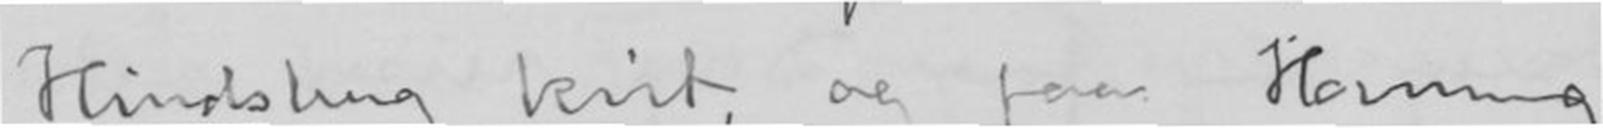Hindsberg kvit, og faa Homung
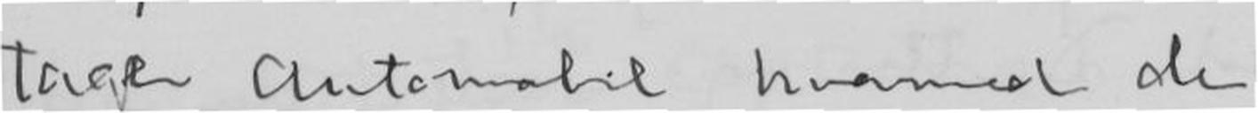tage Automobli hvormed de
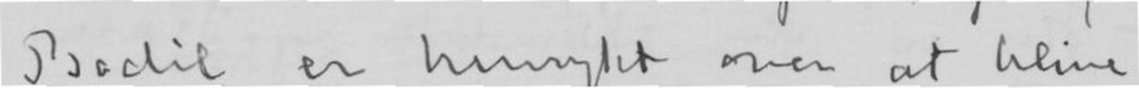Bodil er henrykt over at blive
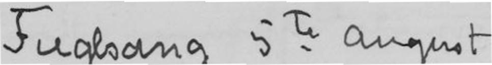Fuglsang 5te August
